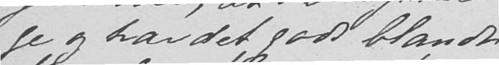ge og har det godt blandt
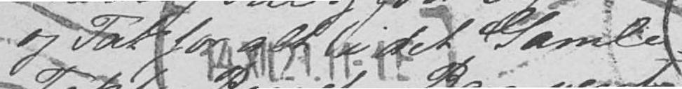og Tak for alt det Gamle.
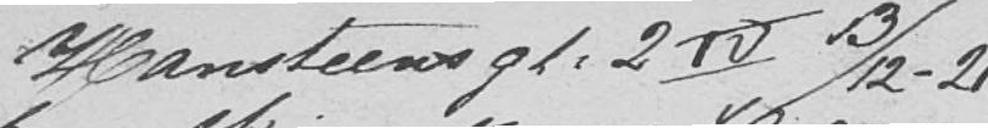Hansteensgt. 2 IV 13/12-21
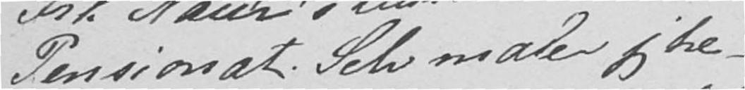Pensionat. Selv maler jeg he-
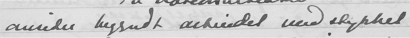omsider begyndt arbeidet med stykket
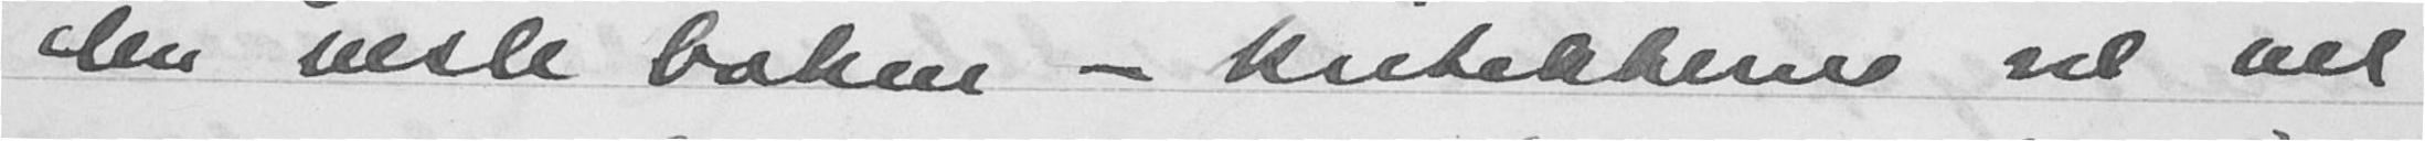den vesle boken - kritikerne vil vel
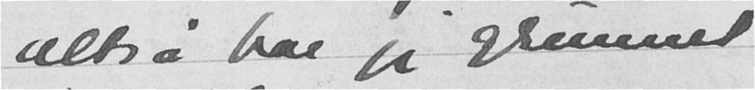altså har jeg grunnet
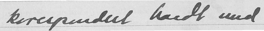korespondert hardt med
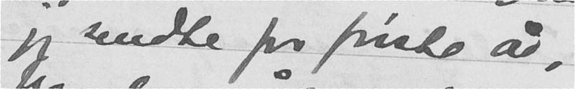vi sendte fra forrige år,
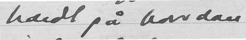hardt på hvordan
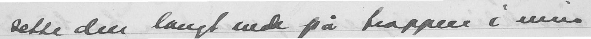sette den langt nede på trappen i min
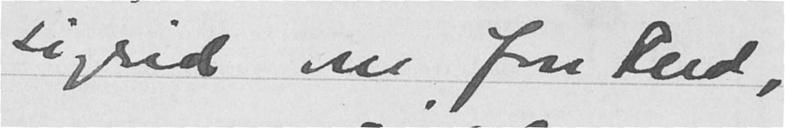Sigrid om fru Frid,
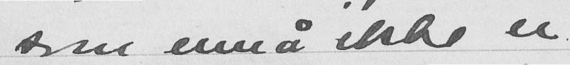som ennå ikke er
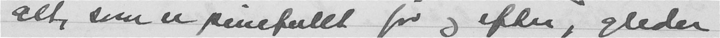alt, som er pinefullt før og efter, gleder
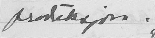produksjon.
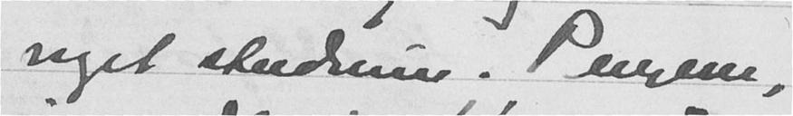noget studium. Pengene,
